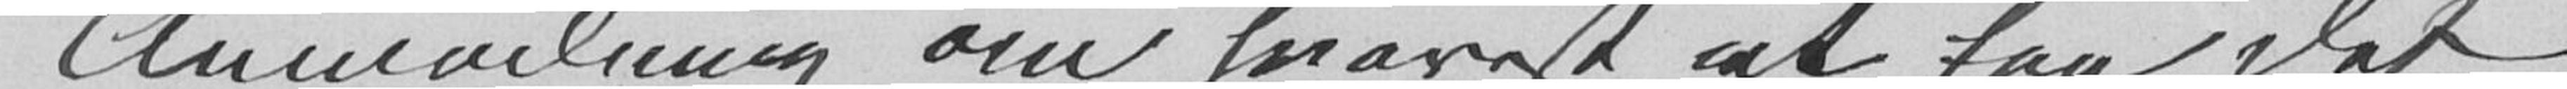Anmodning om snarest at faa det
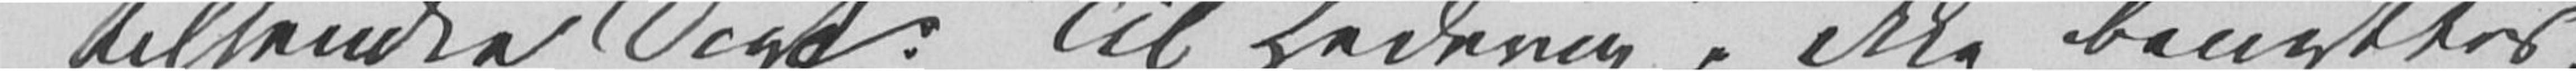tilsendte Digt: «Til Hedevig», ikke benyttes,
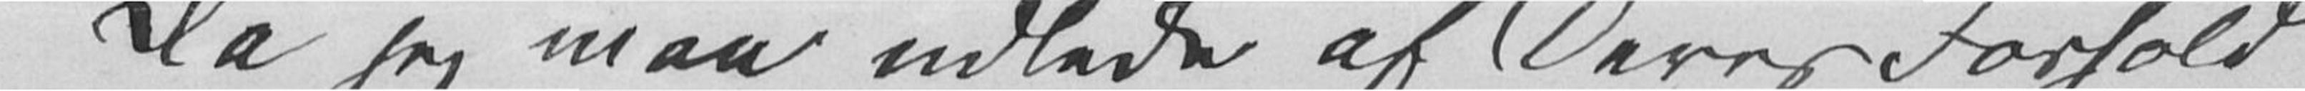Da jeg maa udlede af Deres Forhold
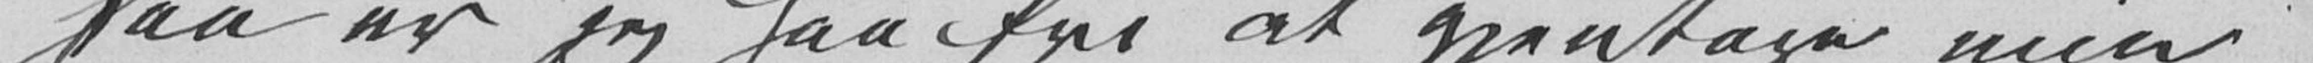saa er jeg saa fri at gjentage min
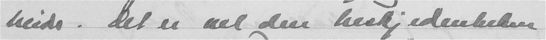beede. Det er vel den beskjedenheten
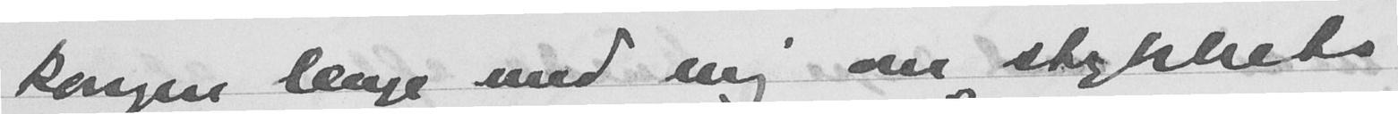kongen lenge med mig om stykkets
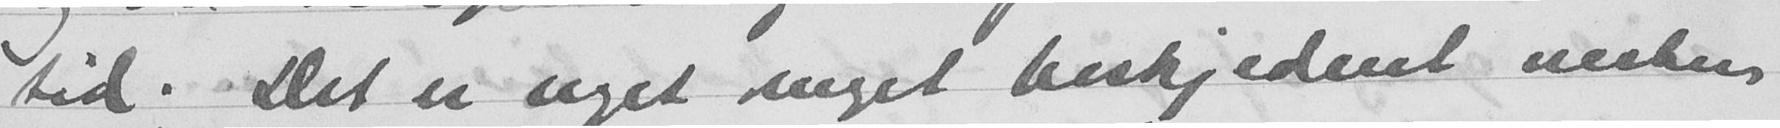tid. Det er noget meget beskjedent nesten
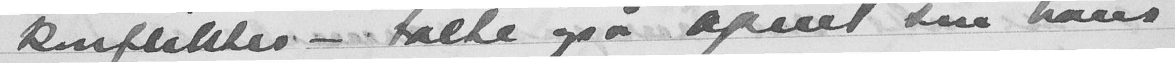konflikter - talte også åpent som hans
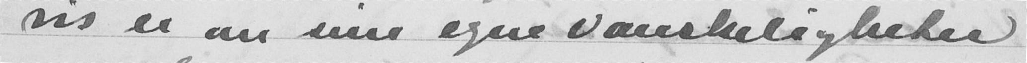vis er om sine egne vanskeligheter
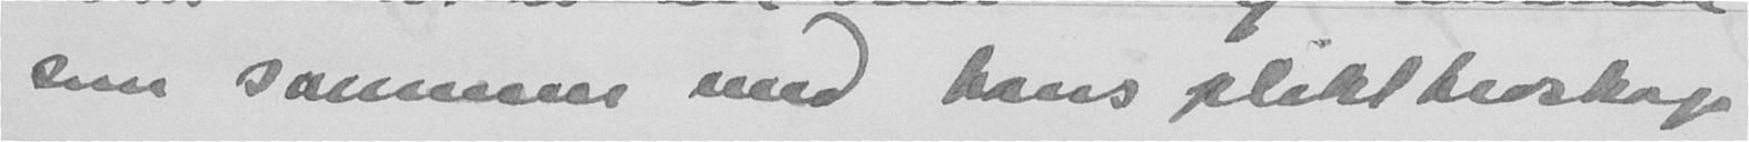som sammen med hans pliktktroskap
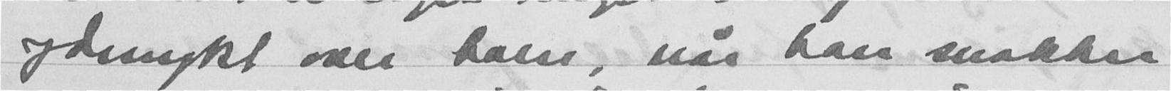ydmykt over ham, når han snakker
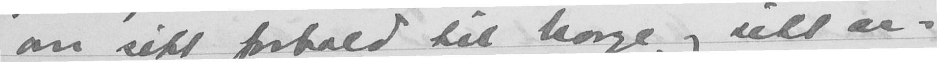om sitt forhold til Norge og sitt ar-
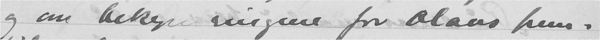og om bekymringene for Olavs frem-
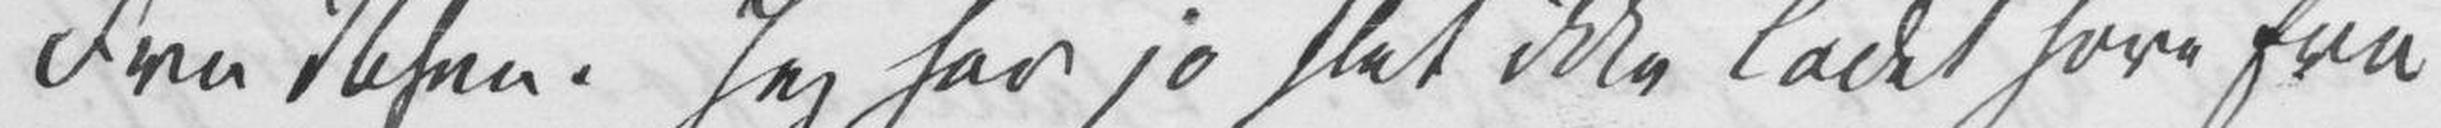Fru Ibsen. Jeg har jo slet ikke ladet høre fra
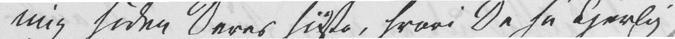mig siden Deres sidste, hvori De så kjerlig
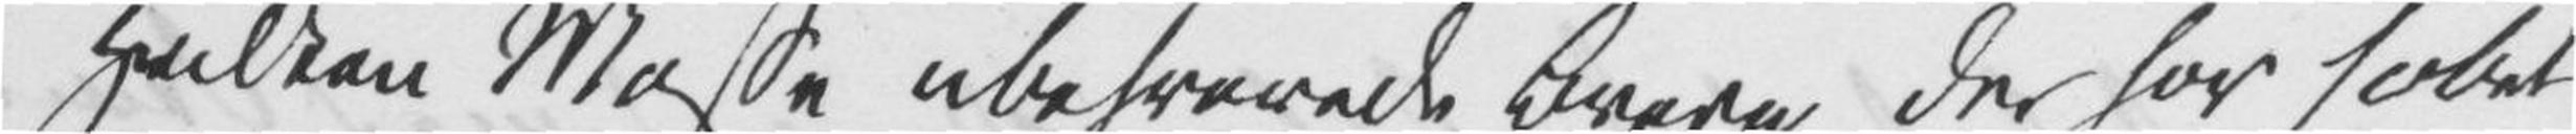Hvilken Masse ubesvarede Breve der har hobet
